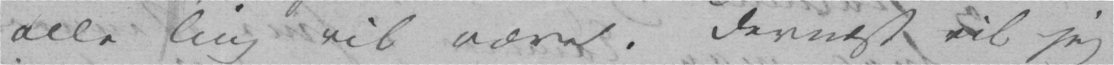alle Ting vil være. Dernæst vil jeg
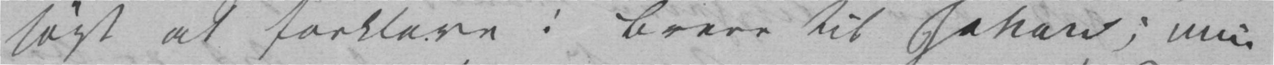søgt at forklare i Breve til Johan; min
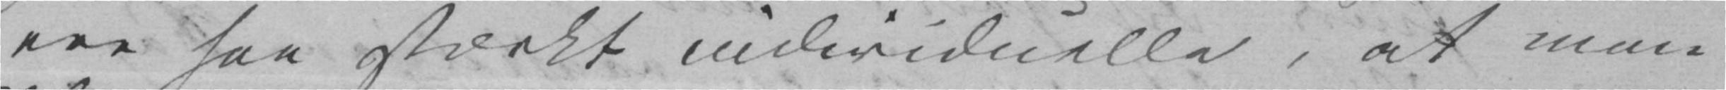ere saa stærkt individuelle, at man
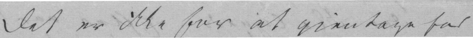Det er ikke for at gjentage for
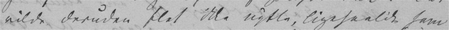vilde desuden slet ikke nytte, ligesaalidt som
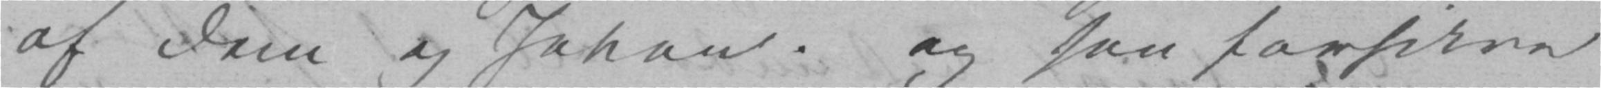af Dem og Johan. og saa forsikre
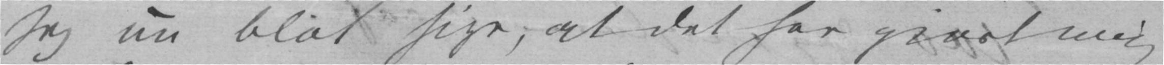jeg nu blot sige, at det har gjort mig
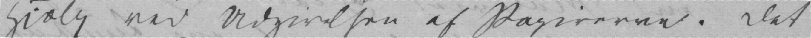Hjælp ved Udgivelsen af Papirerne. Det
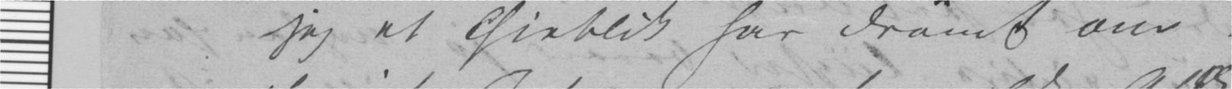jeg et Øieblik har drømt om at kunne over-
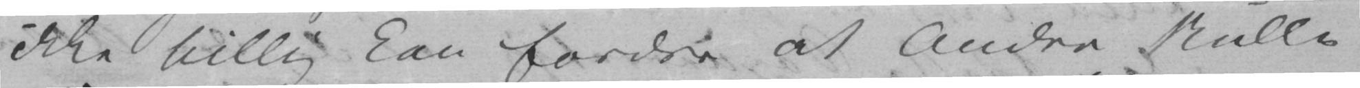ikke billig kan fordre at Andre skulle
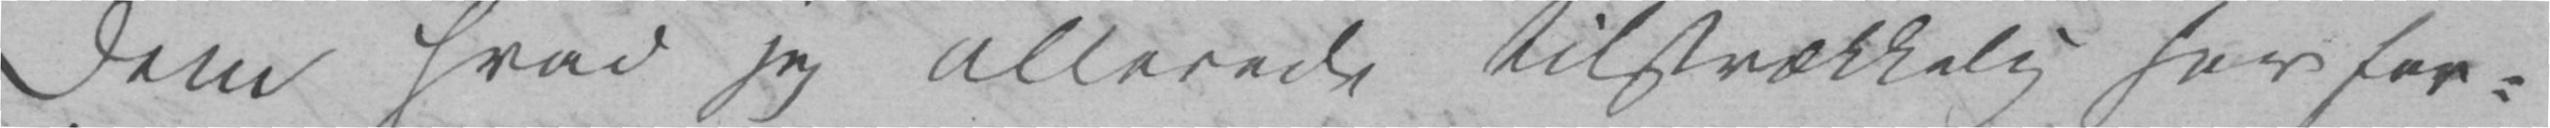Dem hvad jeg allerede tilstrækkelig har for-
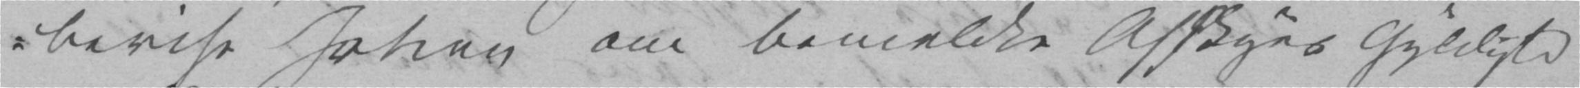bevise Johan om bemeldte Afskyes Gyldighed
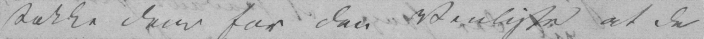takke Dem for den Venlighed at De
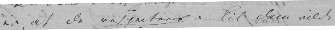er at de respecteres. Til Dem vilde
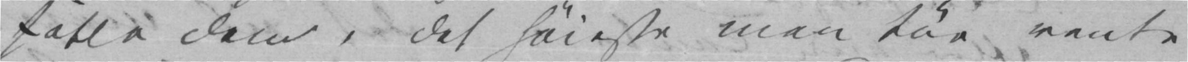fatte dem, det høieste man tør vente
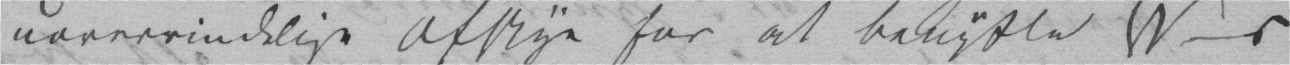uovervindelige Afskye for at benytte W-s
